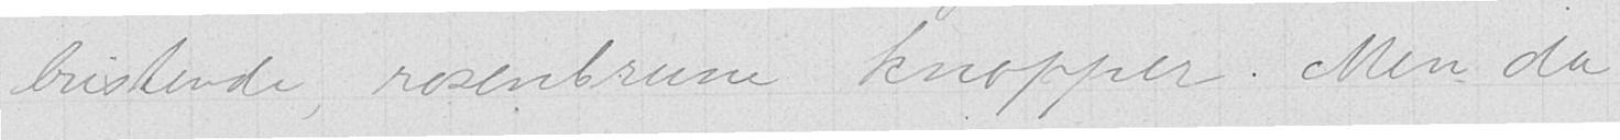bristende, rosenbrune knopper. Men da
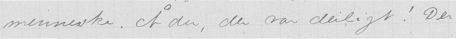menneske. Å du, der var deiligt! Der
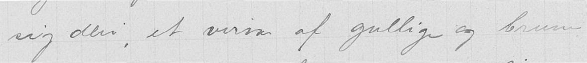sig deri, et virvar af gullige og brune
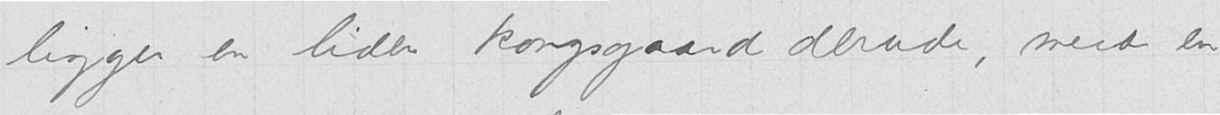ligger en liden kongsgaard derude, med en
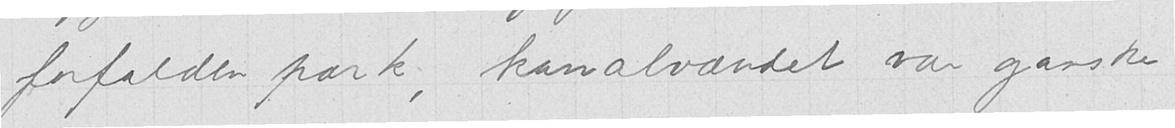forfalden park, kanalvandet var ganske
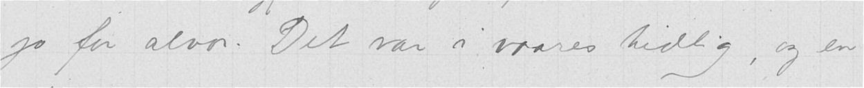jo for alvor. Det var i vaares tidlig, og en
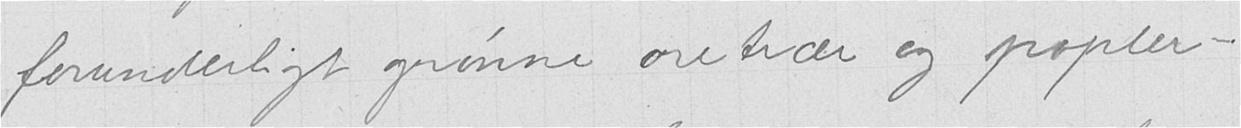forunderligt grønne oretrær og popler -
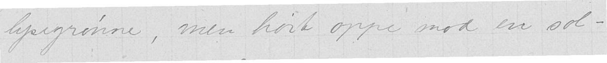lysegrønne, men høit oppe mod en sol-
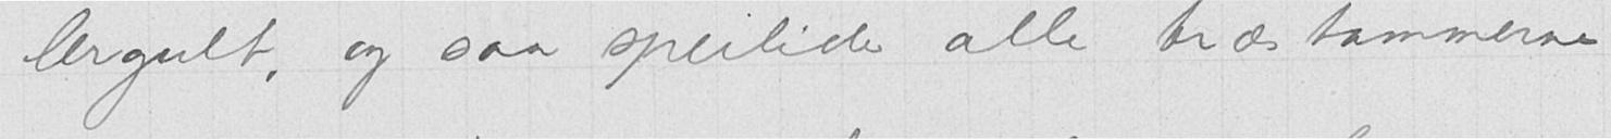lergult, og saa speilede alle træstammerne
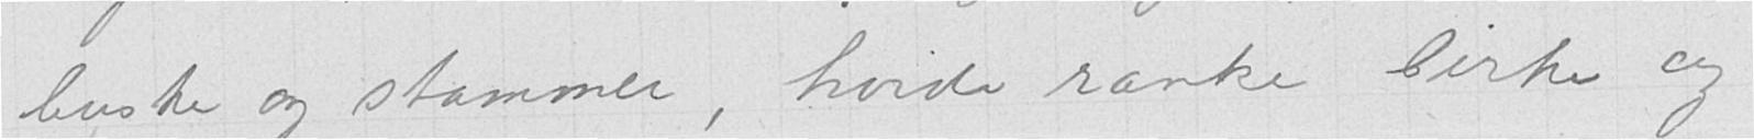buske og stammer, hvide ranke birke og
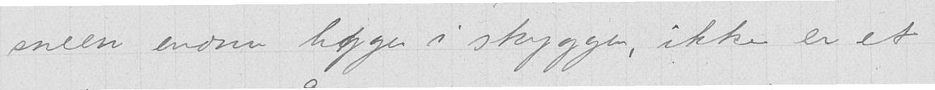sneen endnu ligger i skyggen, ikke er et
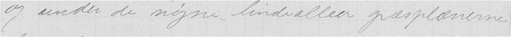og under de nøgne lindealleer græsplænerne
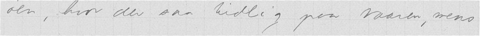øen, hvo der saa tidlig paa Vaaren, mens
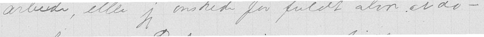arbeide, eller jeg ønskede for fuldt alvor at dø -
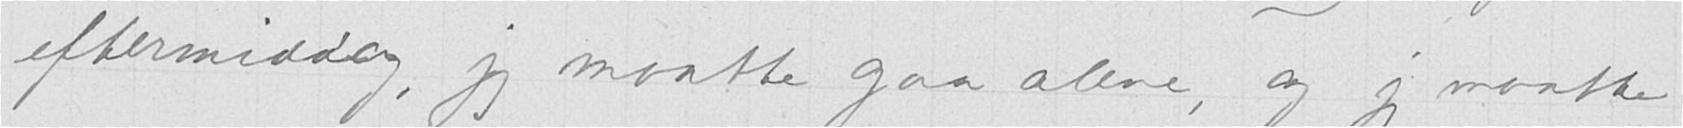eftermiddag, jeg maatte gaa alene, og jeg maatte
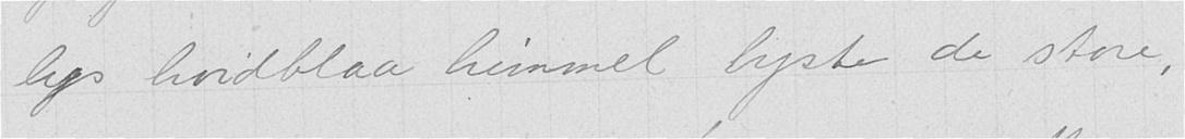lys hvidblaa himmel lyste de store,
